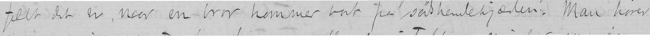fælt det er, naar en bror kommer bort fra sødskendekjæden. Man hører
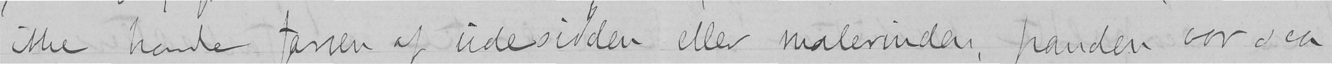ikke havde farven af udesidden eller malerinden, panden var saa
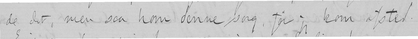de det, men saa kom denne sorg, før jeg kom afsted.
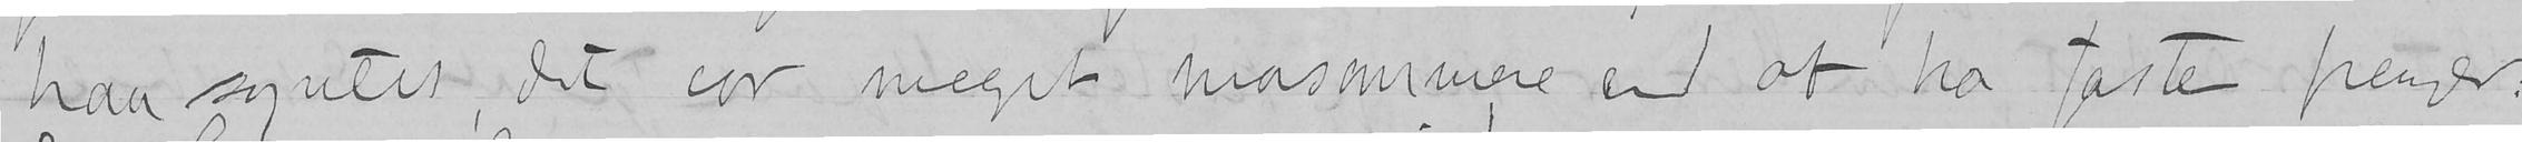han syntes det var meget morsommer end at ha faste penger.
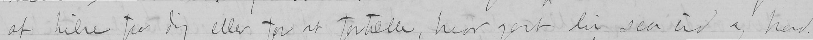at hilse fra dig eller for at fortælle, hvor godt Du saa ud og havd-
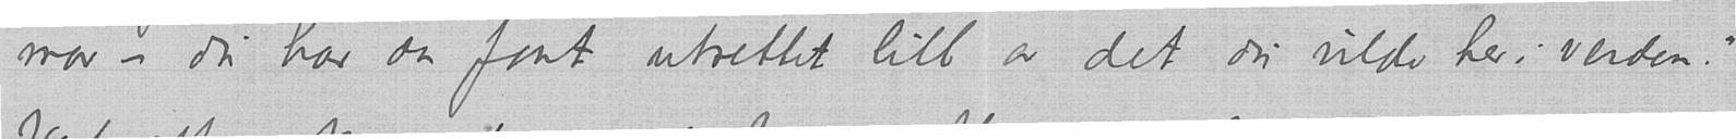mor – du har da faat utrettet litt av det du vilde her i verden."
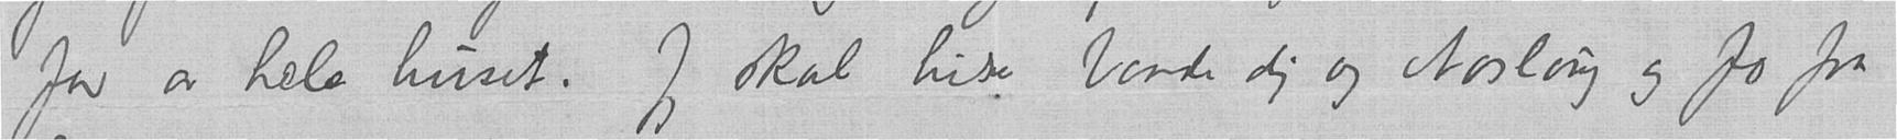for av hele huset. Jeg skal hilse baade dig og Aaslaug og Jo fra
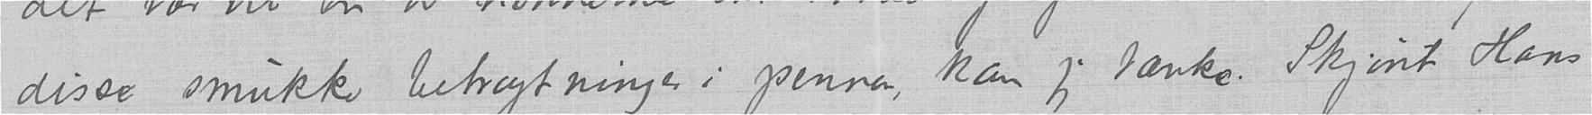disse smukke betragtninger i pennen, kan jeg tænke. Skjønt Hans
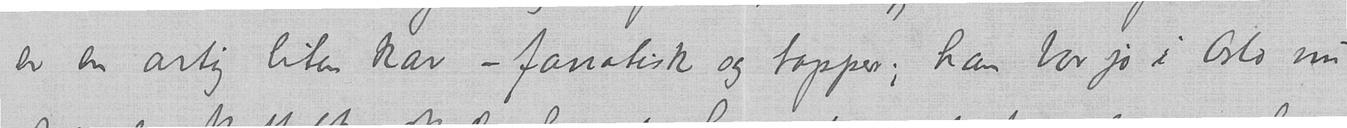er en artig liten kar – fanatisk og tapper; han bor jo i Oslo nu
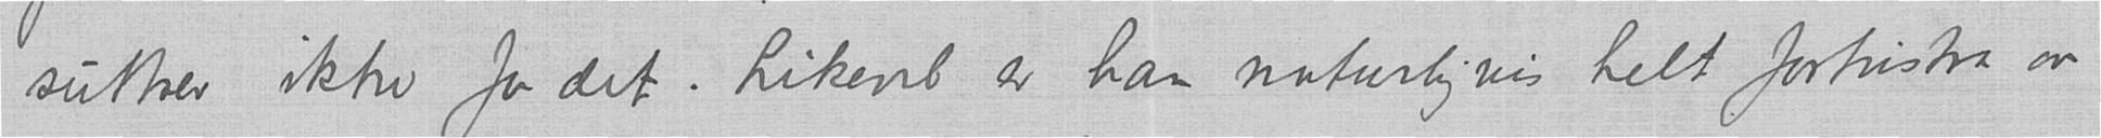suttrer ikke for det. Likevel er han naturligvis helt fortustra av
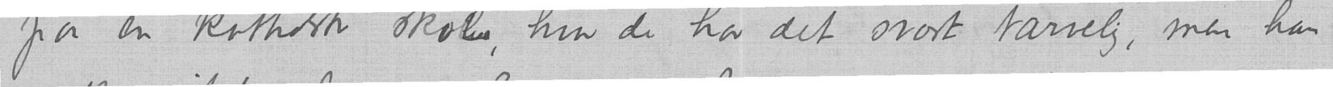paa en katholsk skole, hvor de har det svært tarvelig, men han
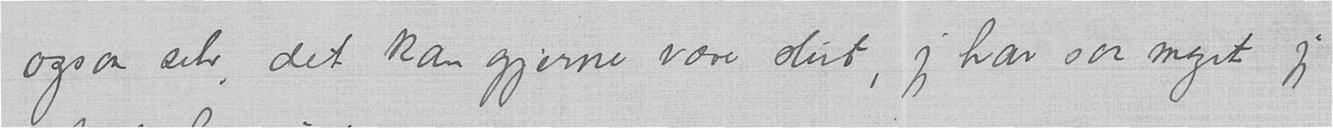ogsaa selv, det kan gjerne være slut, jeg har saa meget jeg
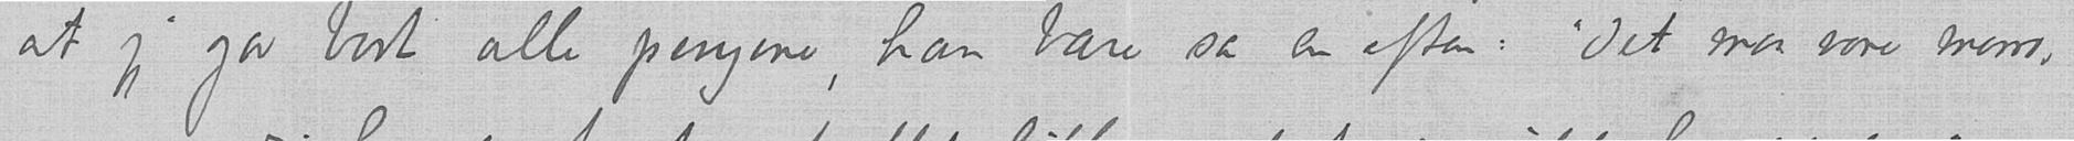at jeg gav bort alle pengene, han bare sa en aften: "Det maa være morro,
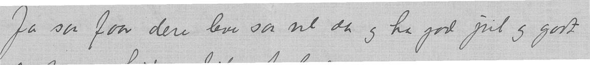Ja saa faar dere leve saa vel da og ha god jul og godt
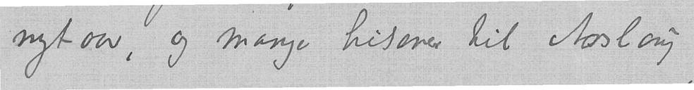nytaar, og mange hilsener til Aaslaug
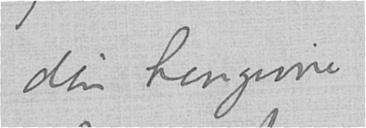din hengivne
